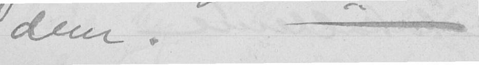dem. -
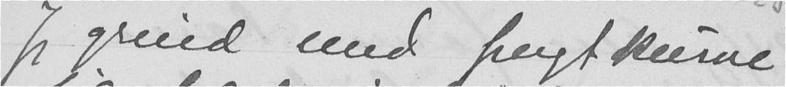Jeg greied med frugtkurve
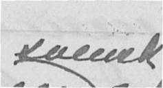svensk
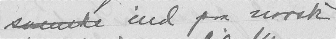svenske ind paa norsk
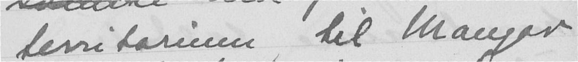territorium, til Mangor
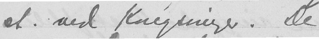st. ved Kongsvinger. De
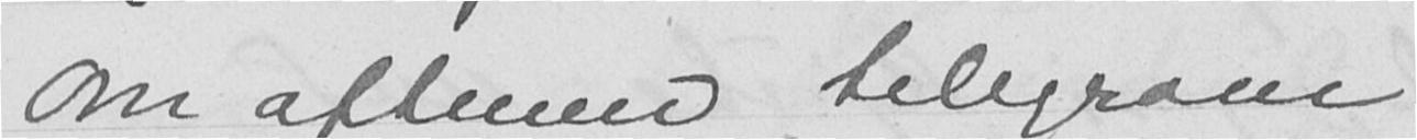Om aftenen telegram
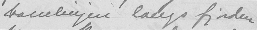banelinjen langs fjorden
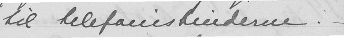til telefonistinderne. -
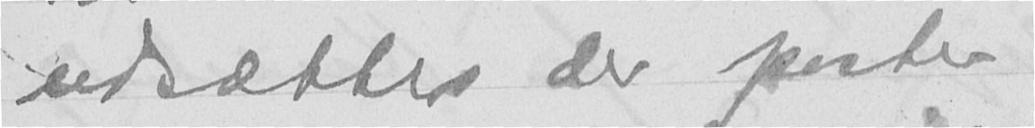udsættes der poster
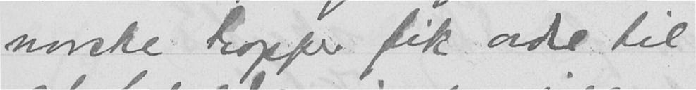norske tropper fik ordre til
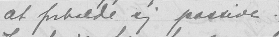at forholde sig passive.
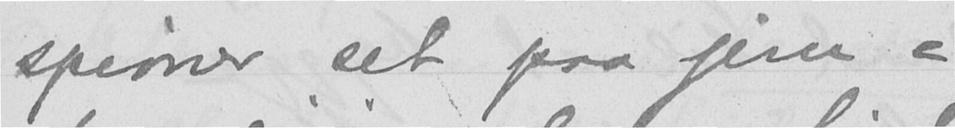spioner set paa jern-
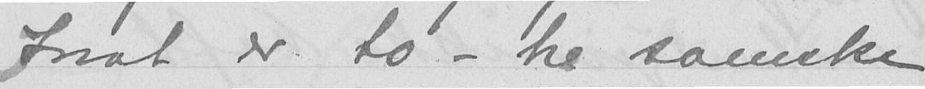Inat er to-tre svenske
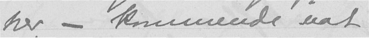her - kommende nat
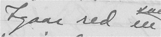Igaar red en
